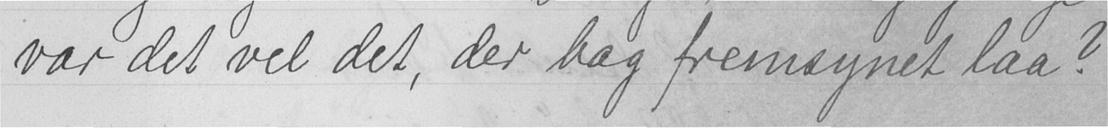var det vel det, der bag fremsynet laa?
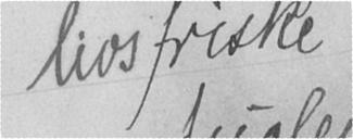livsfriske
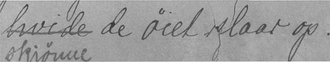hvide de øiet slaar op.
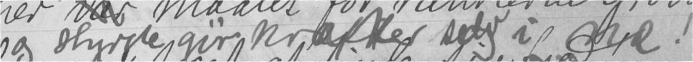g styrke gir kræfter selv i ne!
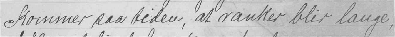Kommer saa tiden, at ranker blir lange,
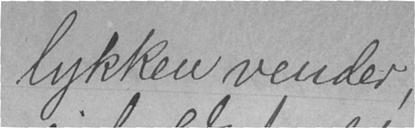lykken vender,
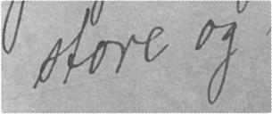store og
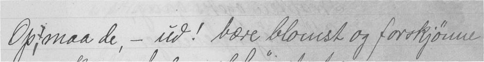Op, maa de, - ud! bære blomst og forskjønne
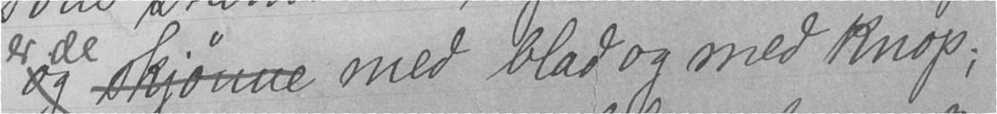og skjønne med blad og med knop;
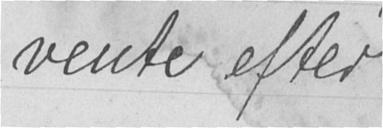vente efter
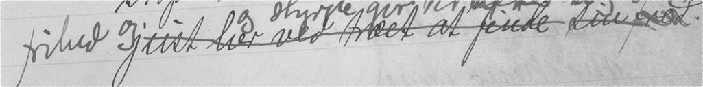frihed og just her ved træet at finde sin fred.
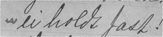- ei holdt fast!
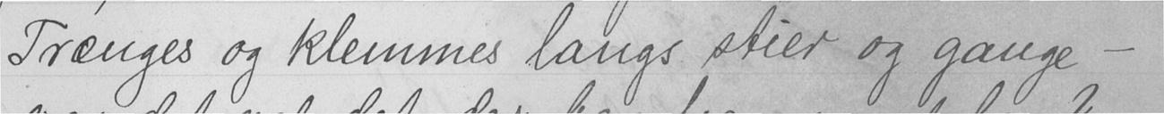Trænges og klemmes langs stier og gange -
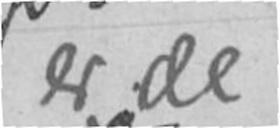er de
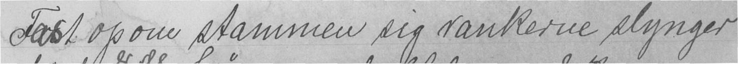Fast op om stammen sig rankerne slynger,
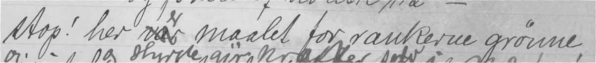stop! her var er maalet for rankerne grønne,
